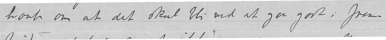haab om at det skal bli ved at gaa godt i frem-
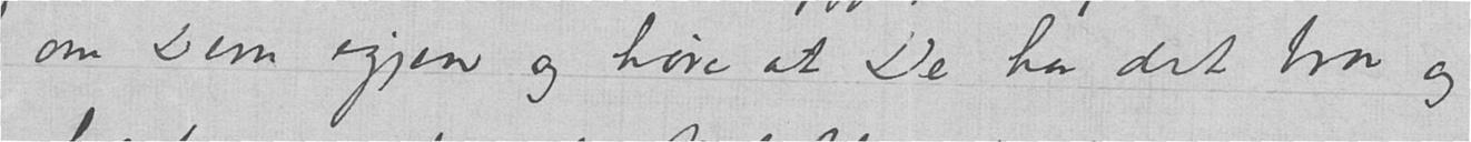om Dem igjen og høre at De har det bra og
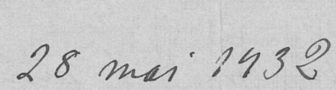28. mai 1932
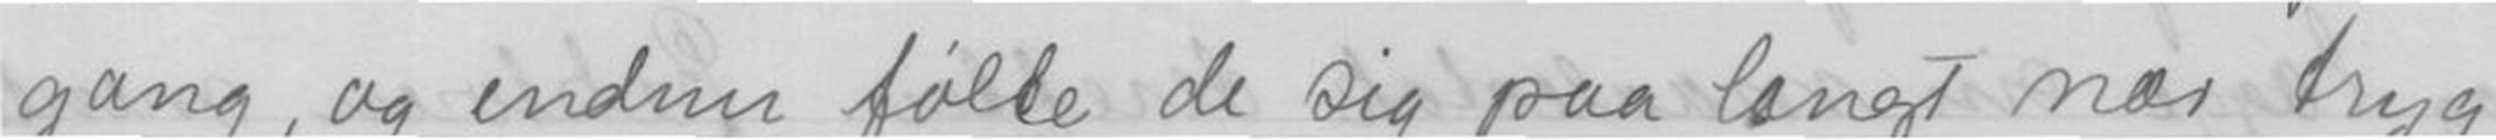gang, og endnu følte de sig paa langt mær tryg
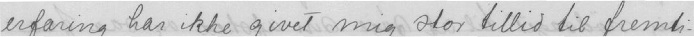erfaring har ikke givet mig stor tillid til fremti-
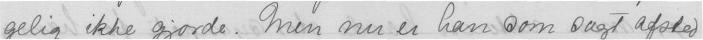gelig ikke gjorde. Men nu er han som sagt afsted
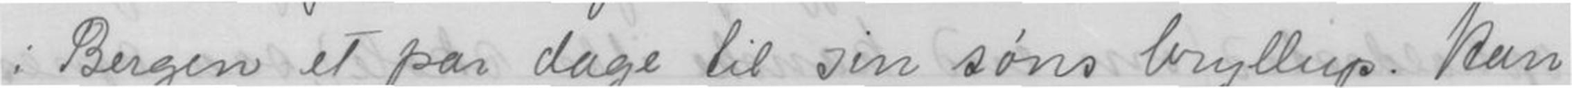i Bergen et par dage til sin søns bryllup. Han
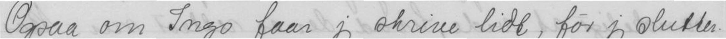Ogsaa om Ingo faar jeg skrive lidt, før jeg slutter.
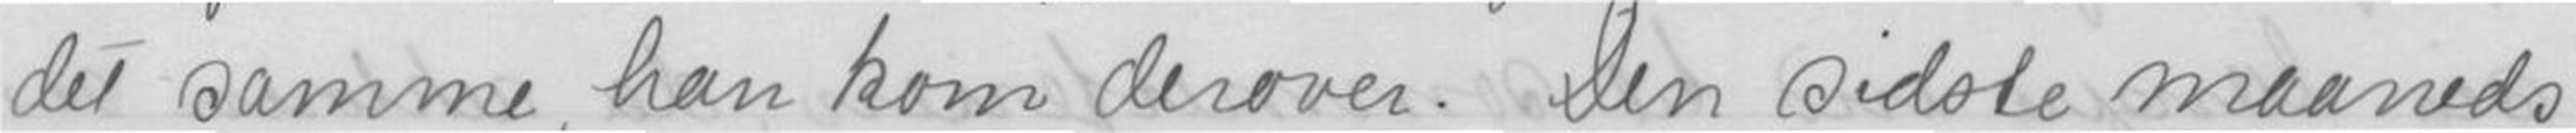det samme, han kom derover. Den sidste maaneds
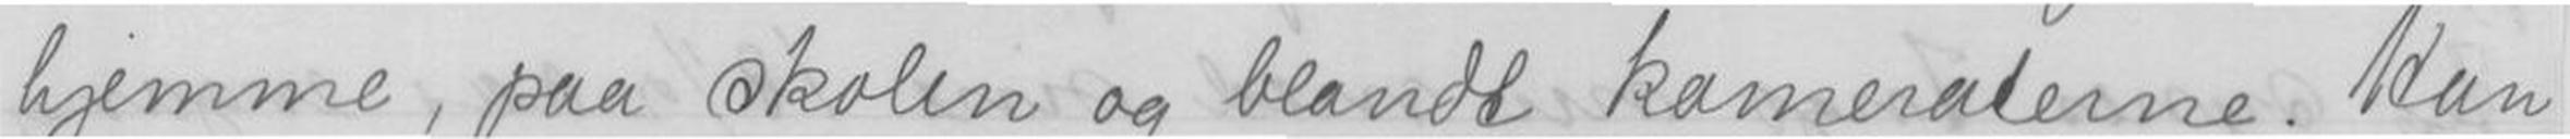hjemme, paa skolen og blandt kameraterne. Han
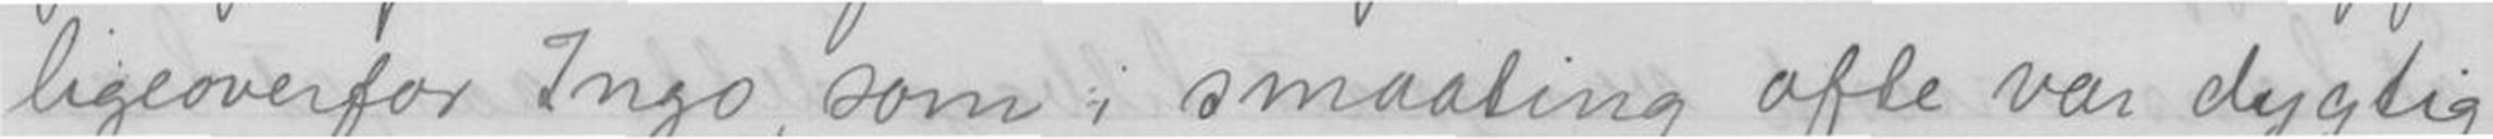ligeoverfor Ingo, som i smaating ofte var dygtig
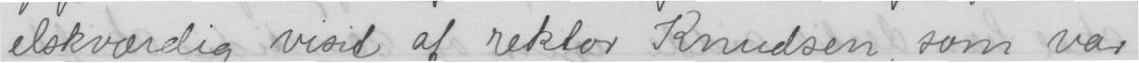elskværdig visit af rektor Knudsen, som var
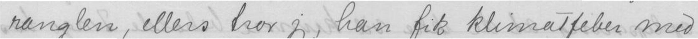ranglen, ellers tror jeg, han fik klimatfeber med
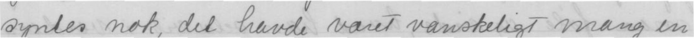syntes nok, det havde været vanskeligt mang en
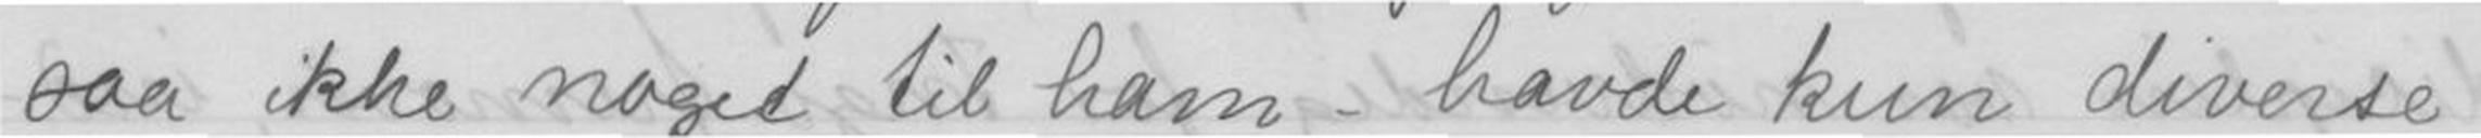saa ikke noget til ham _ havde kun diverse
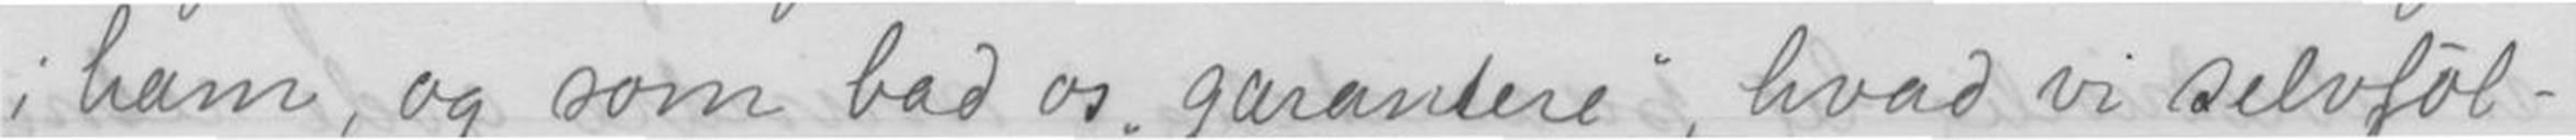i ham, og som bad os garantere, hvad vi selvføl-
